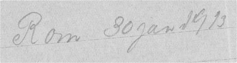Rom 30 jan 1913
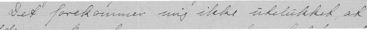Det forekommer mig ikke utelukket, at
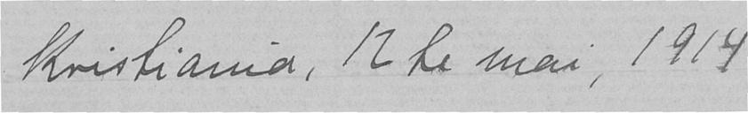Kristiania, 12te mai, 1914
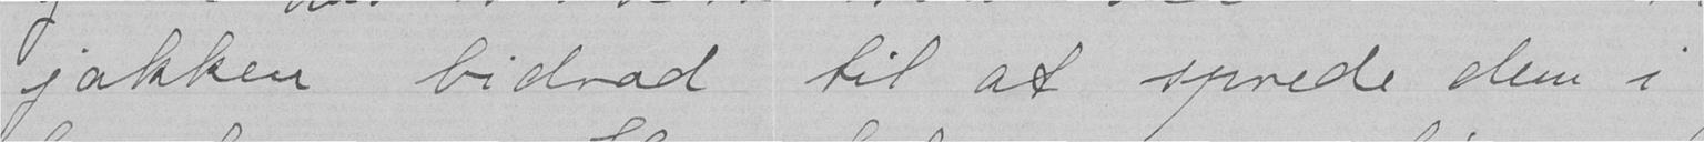jakken bidrad til at sprede dem i
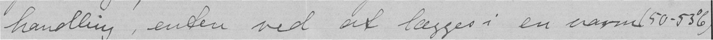handling, enten ved at lægges i en varme (50-53°C)
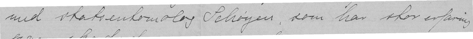med Statsentomolog Schøyen, som har stor erfaring
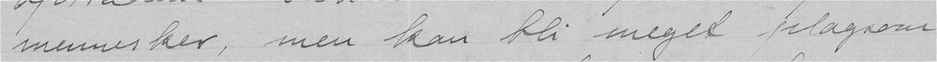mennesker, men kan bli meget plagsom
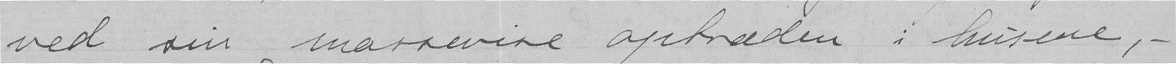ved sin massevise optræden i husene, -
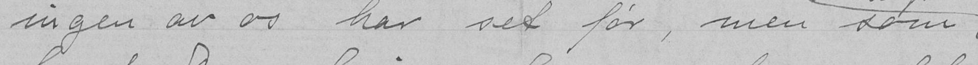ingen av os har set før, men som
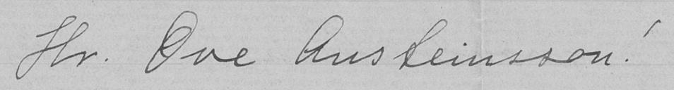Hr. Ove Ansteinsson!
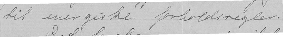til energiske forholdsregler.
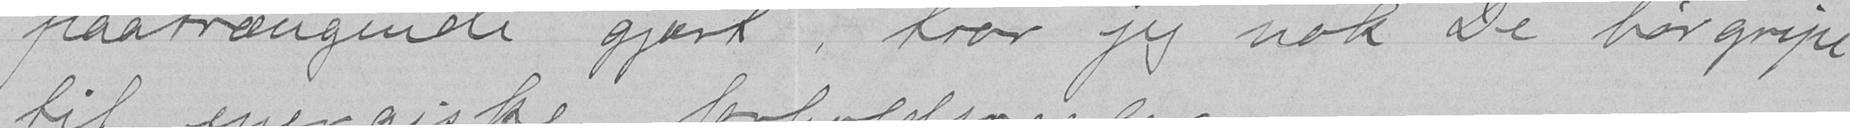paatrængende gjæst, tror jeg nok De bør gripe
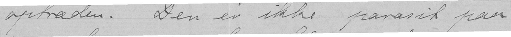optræden. Den er ikke pavasit paa
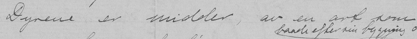Dyrene er midder, av en art som
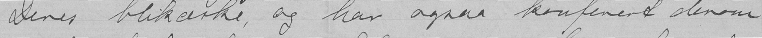Deres blikæske, og har ogsaa konferert derom
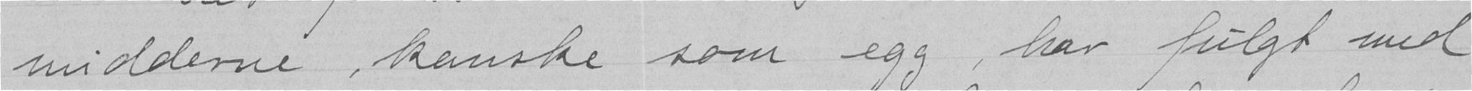midderne, kanske som egg, har fulgt med
